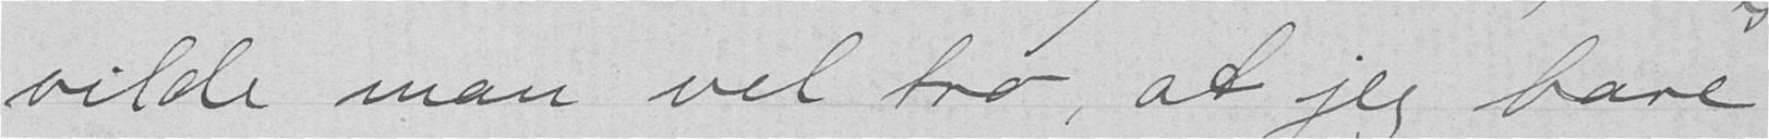vilde man vel tro, at jeg bare
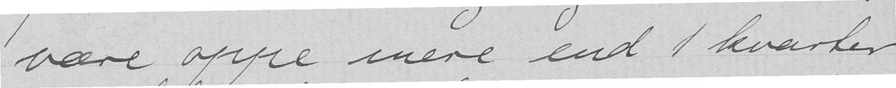være oppe mere end 1 kvarter
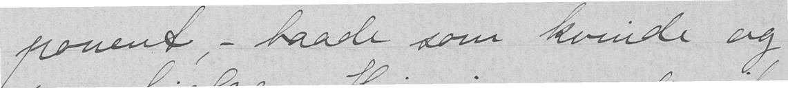ponent, - baade som kvinde og
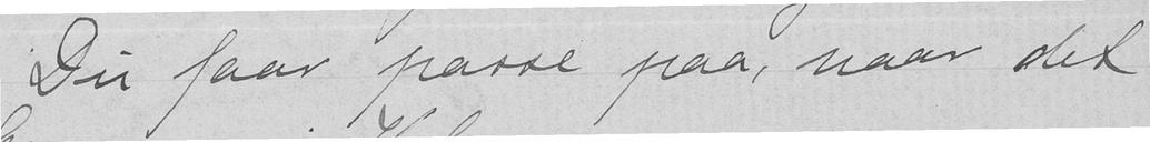Du faar passe paa, naar det
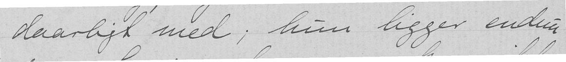daarligt med; hun ligger endnu
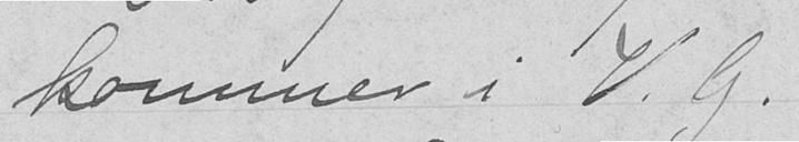kommer i V. G.
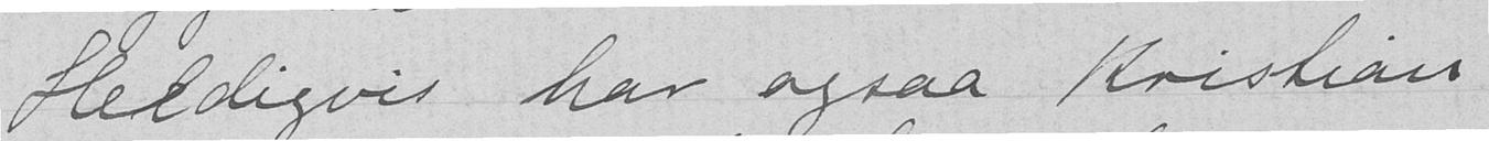Heldigvis har ogsaa Kristian
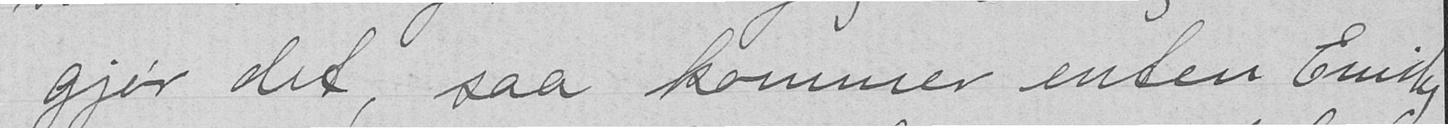gjør det, saa kommer enten Emily
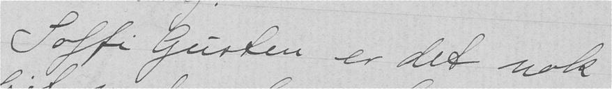Soffi Gusten er det nok
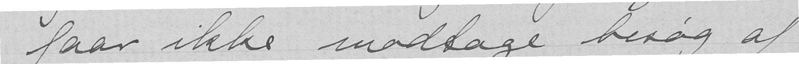faar ikke modtage besøg af
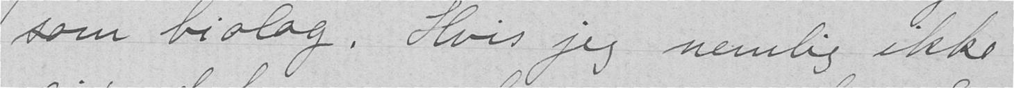som biolog. Hvis jeg nemlig ikke
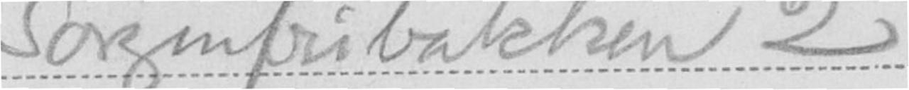Sorgenfribakken 2
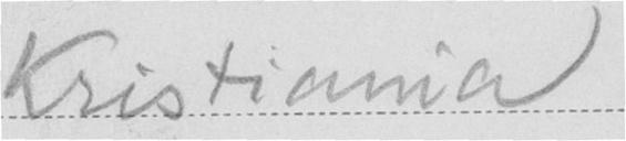Kristiania
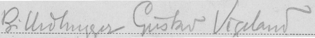Billedhugger Gustav Vigeland
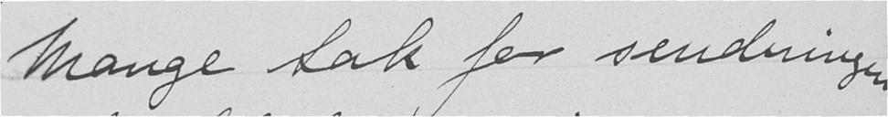Mange tak for sendningen
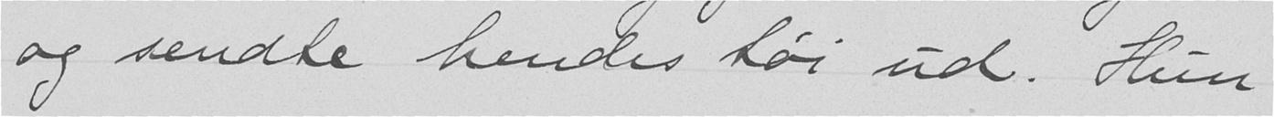og sendte hendes tøi ud. Hun
